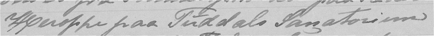Heroppe paa Tuddals Sanatorium
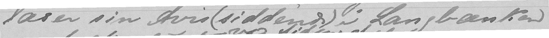læser sin Avis (siddende) i Langbænken
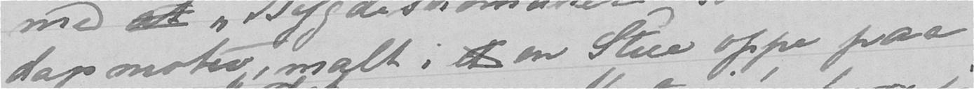dagsmotiv, malt i et en Stue oppe paa
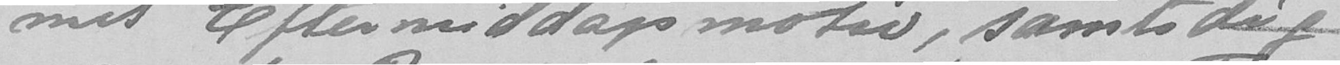mit Eftermiddagsmotiv, samtidig
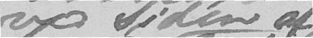ved siden af
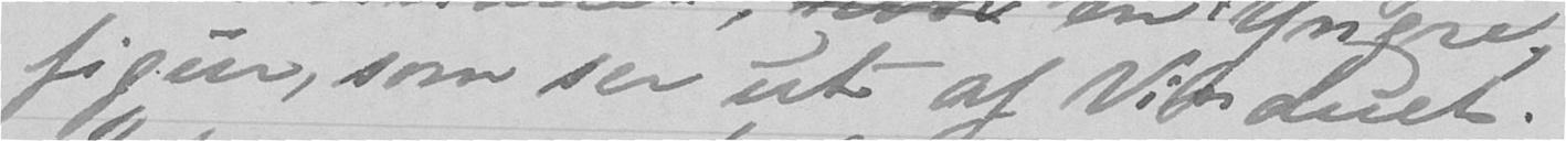figur, som ser ut af Vinduet.
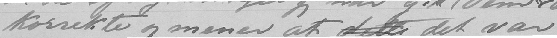korrekte og mener at dette det var
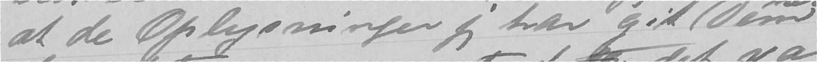at de Oplysninger jeg har git Dem
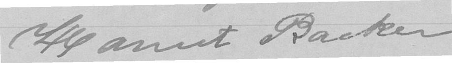Harriet Backer
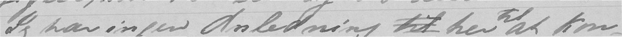Jeg har ingen Anledning til her til at kon-
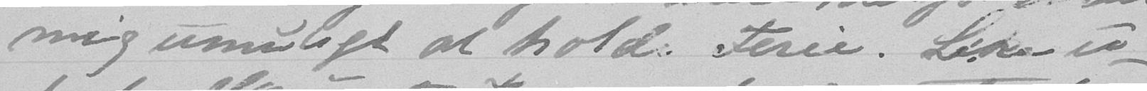mig umuligt at holde Ferie. Like u-
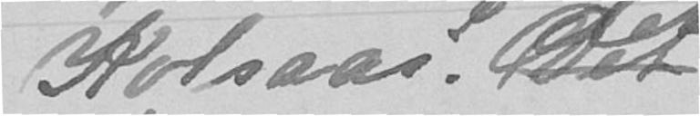Kolsaas. Det
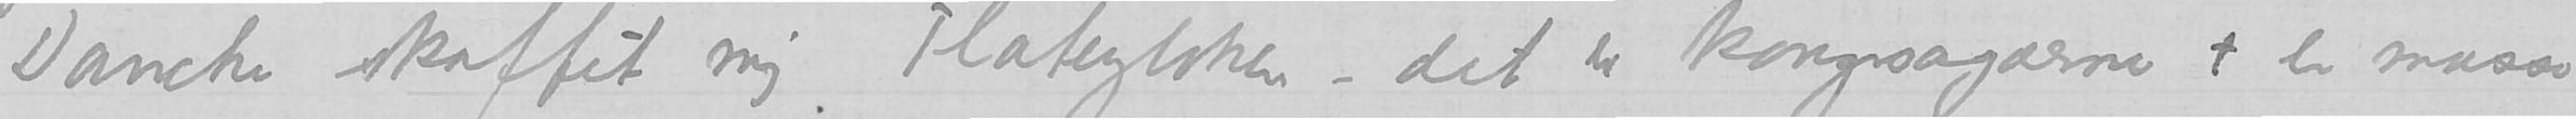Danche skaffet mig Flateyboken- det er kongesagaerne + en masse
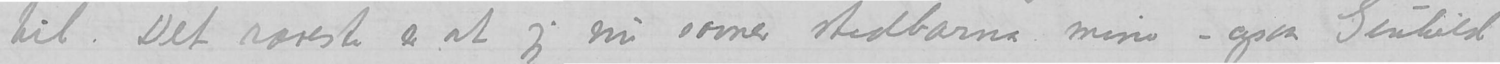til.  Det rareste er at jeg nu savner stedbarna mine – ogsaa Gundhild
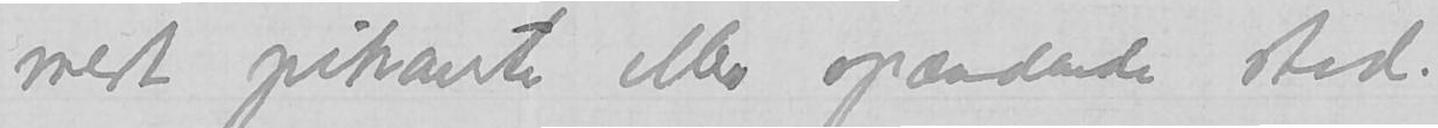mest pikante eller spændende sted.
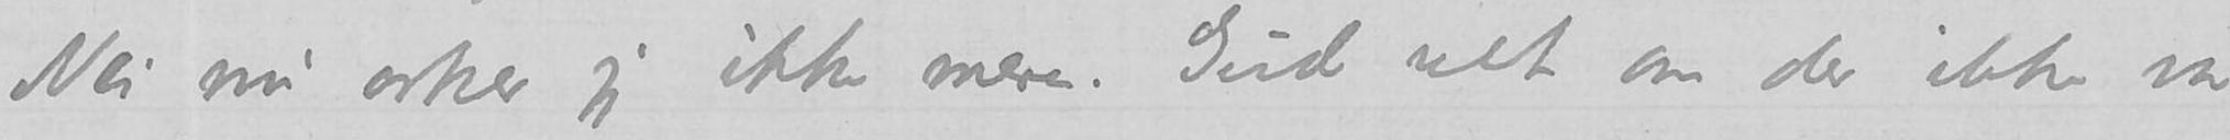Nei nu orker jeg ikke mere. Gud vet om der ikke var
